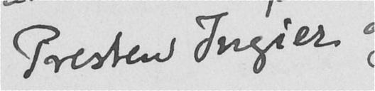Presten Ingier
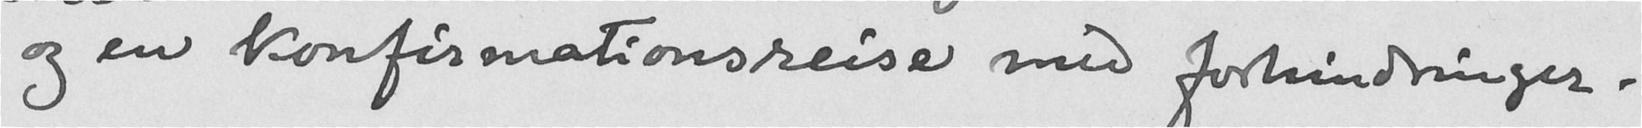og en konfirmationsreise med forhindringer.
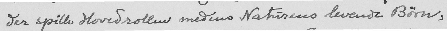der spille Hovedrollen medens Naturens levende Børn,
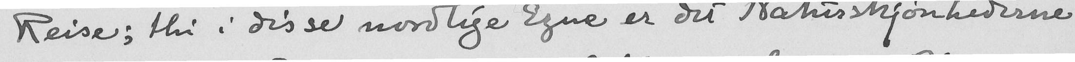Reise, thi i disse nordlige Egne er det Naturskjønhederne
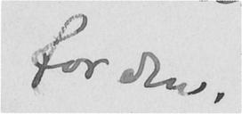for den."
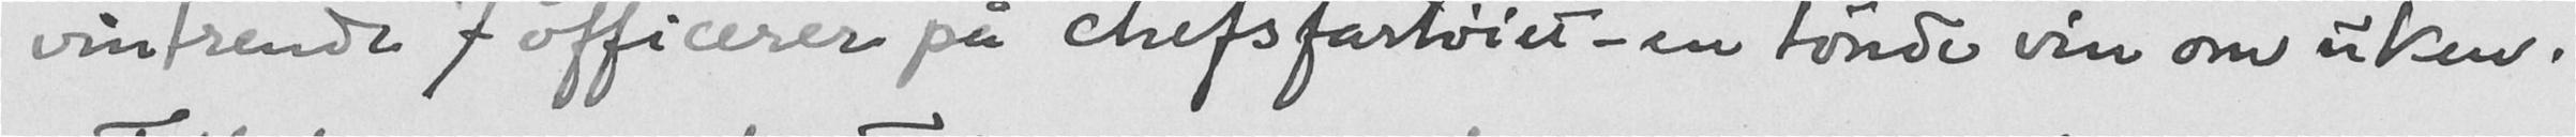vintrende 7 officerer på chefsfartøiet - en tønde vin om uken.
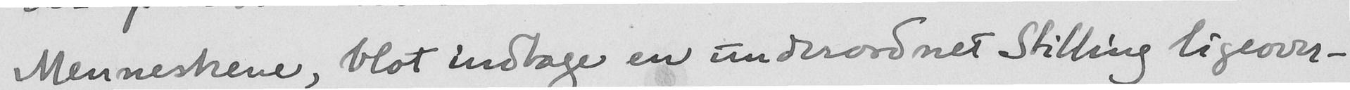Menneskene, blot indtage en underordnet stilling ligeover-
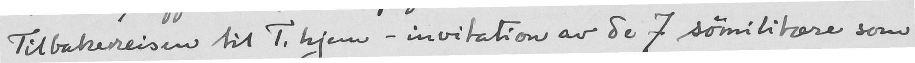Tilbakereisen til T.hjem - invitation av de 7 sømilitære som
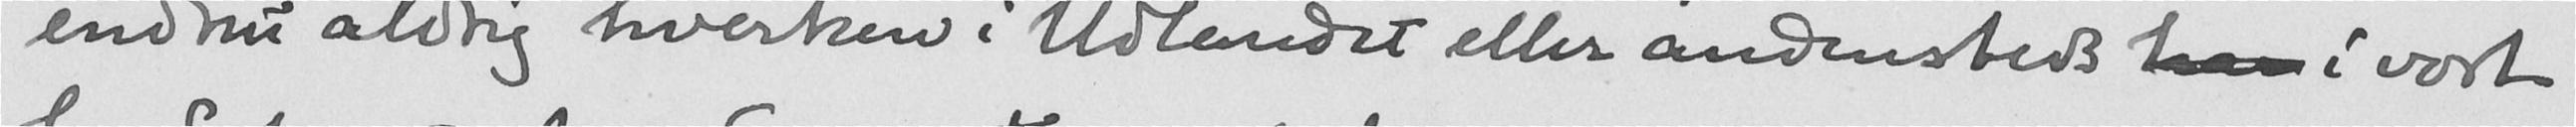endnu aldrig hverken i Udlandet eller andensteds hæn i vort
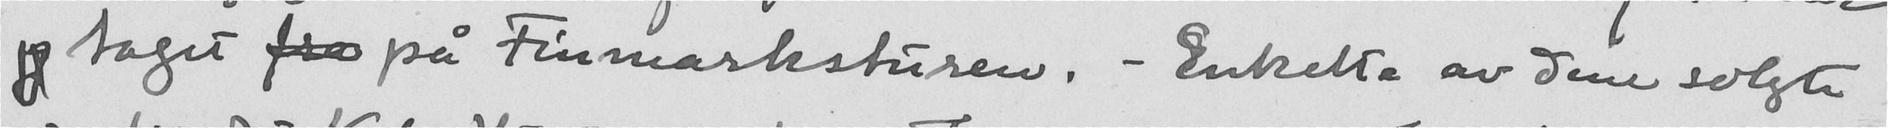og taget fra på Finmarksturen. - Enkelte av dine solgte
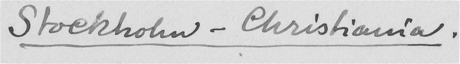Stockholm - Christiania.
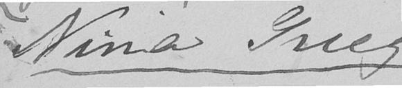Nina Grieg
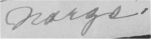Norge.
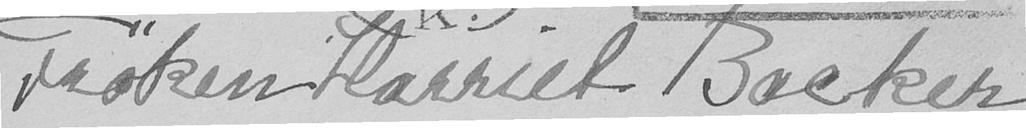Frøken Harriet Backer
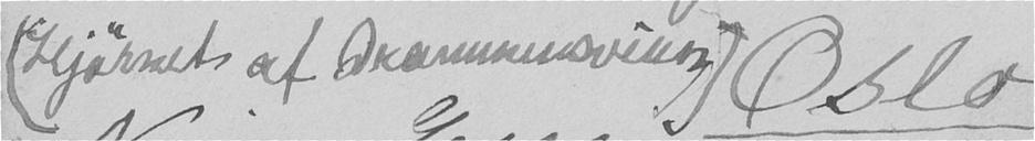(Hjørnet af Drammenveien) Oslo
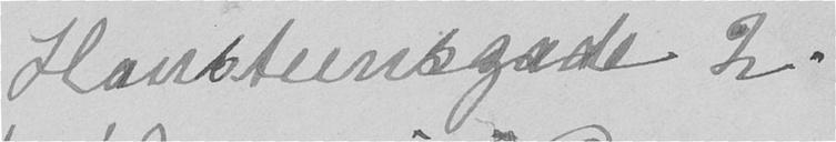Hansteensgade 2.
